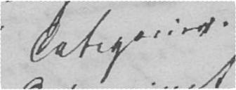Categorier.
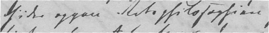sider opgav Retsphilosophien
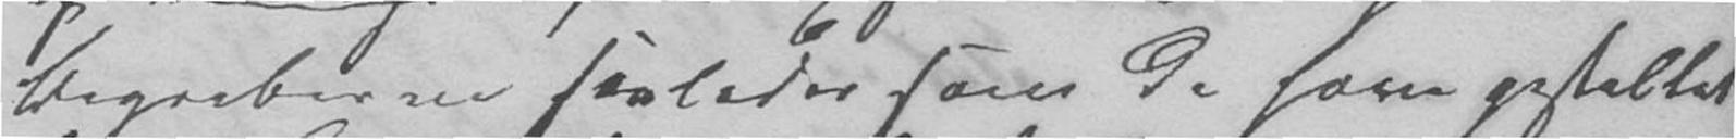Begreberne saaledes som de have gestaltet
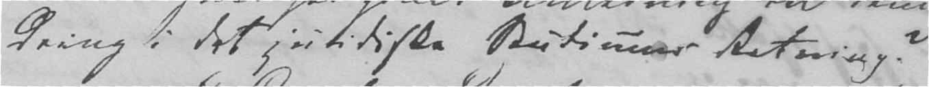dring i det juridiske Studiums Retning?
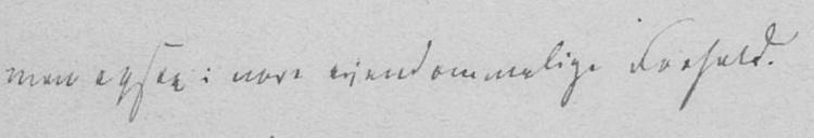men ogsaa i vore eiendommelige Forhold.
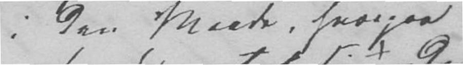i den Maade, hvorpaa
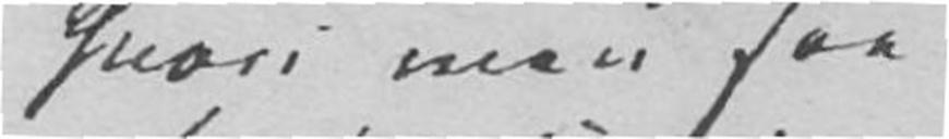hvori man saa
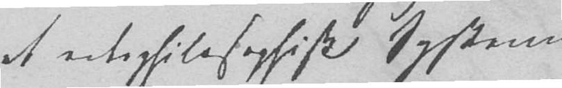et philosophisk System
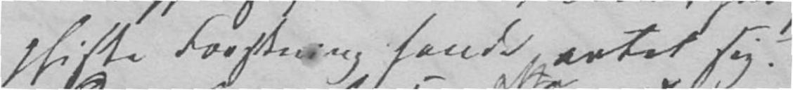phiske Forskning havde artet sig
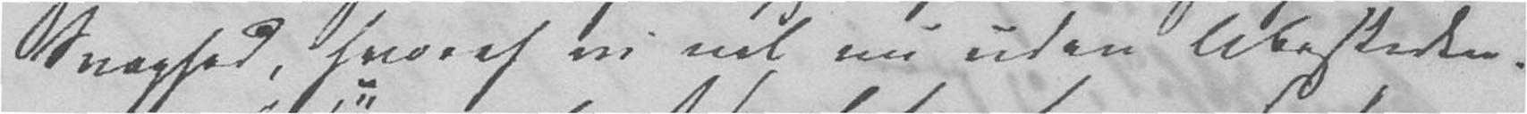Svaghed, hvoraf vi vel nu uden Ubeskeden-
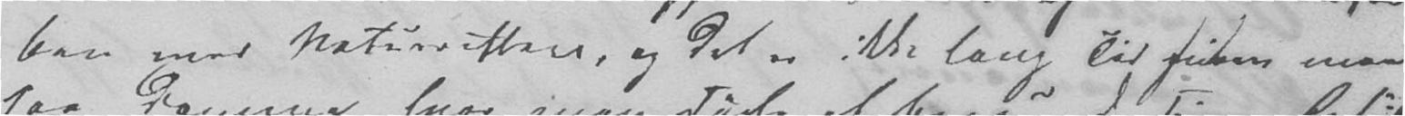ben med Naturretten, og det er ikke lang Tid siden man
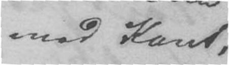med Kant,
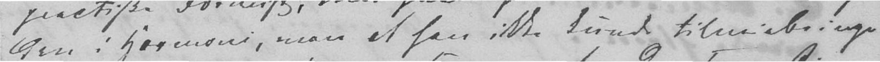den i Harmoni, men at han ikke kunde tilveiebringe
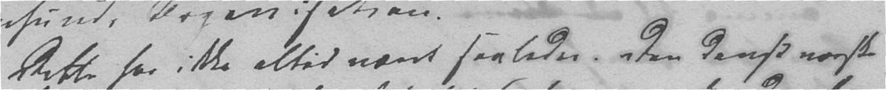Dette har ikke altid været saaledes. Den dansk norske
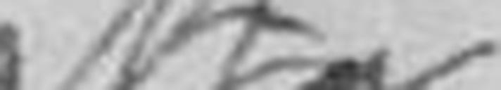ofte
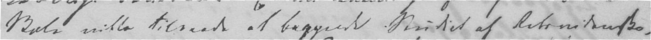Skole ville tilraade at begynde Studiet af Retsvidenska-
